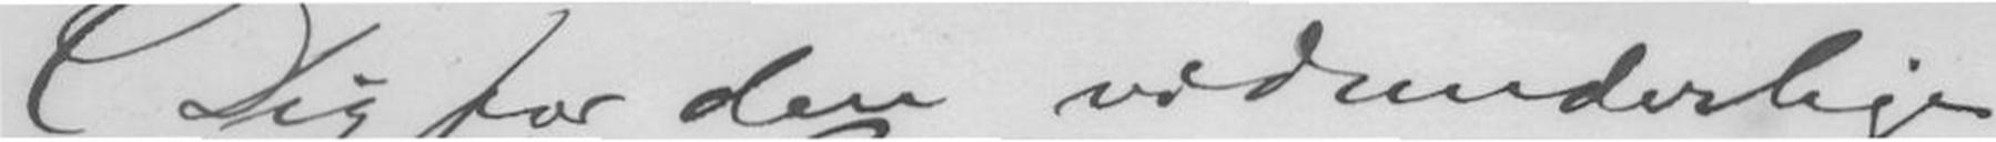Dig for den vidunderlige
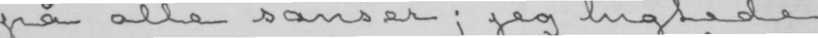på alle sanser; jeg lugtede
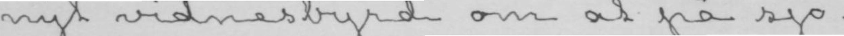nyt vidnesbyrd om at på sjø-
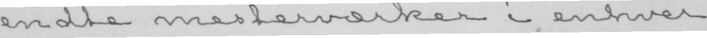endte mesterværker i enhver
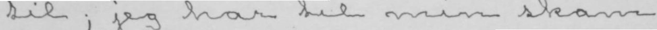til; jeg har til min skam
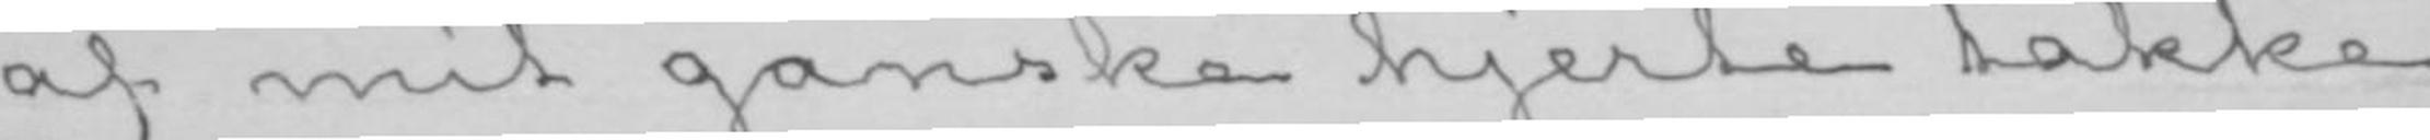af mit ganske hjerte takke
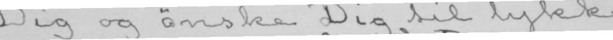Dig og ønske Dig til lykke
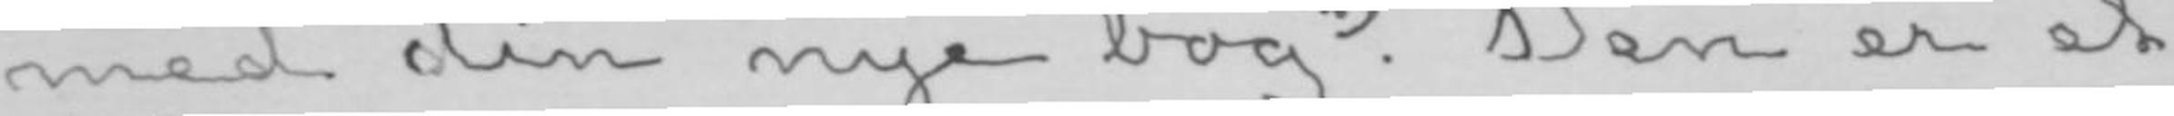med din nye bog. Den er et
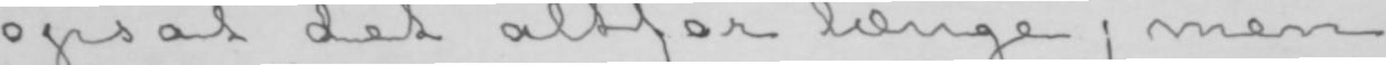opsat det altfor længe; men
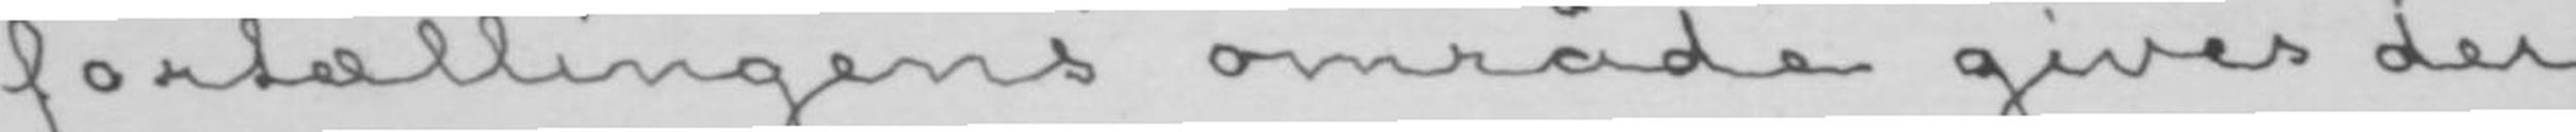fortællingens område gives der
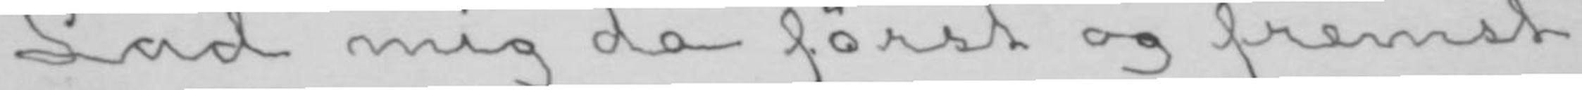Lad mig da først og fremst
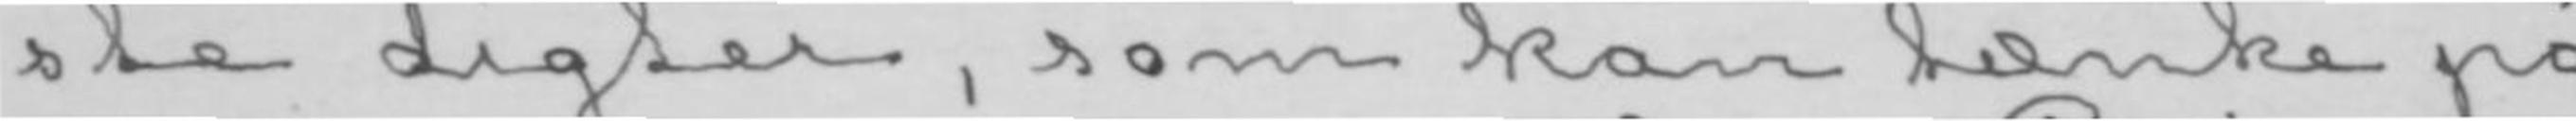ste digter, som kan tænke på
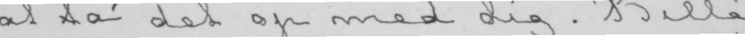at ta&rapo; det op med dig. Bille-
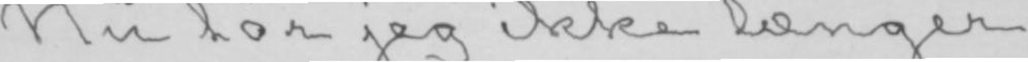Nu tør jeg ikke længer
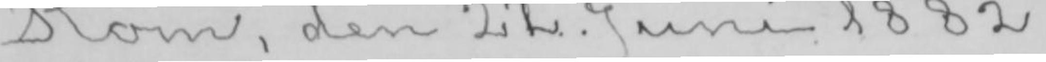Rom, den 22. Juni 1882.
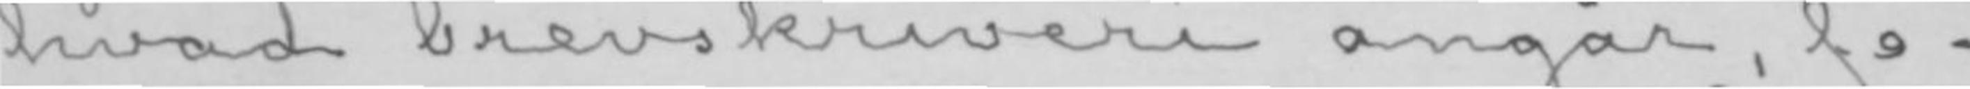hvad brevskriveri angår, fø-
